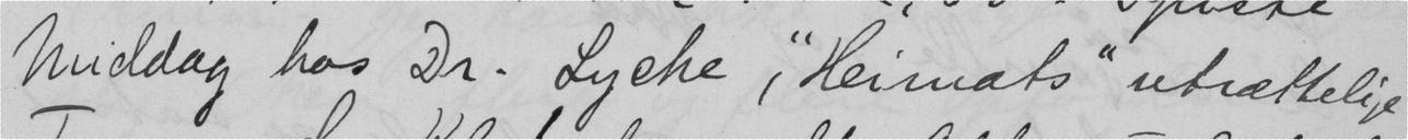middag hos Dr. Lyche, "Heimats" utrættelige
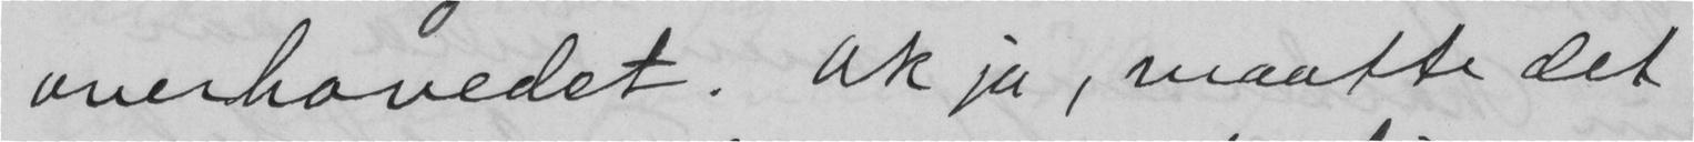overhovedet. Ak ja, maatte det
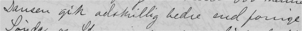Dansen gik adskillig bedre end forrige
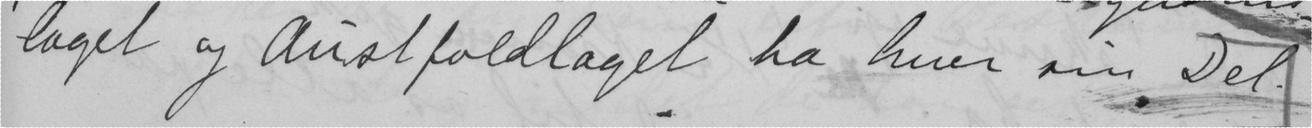laget og Austfoldlaget ha hver sin Del.
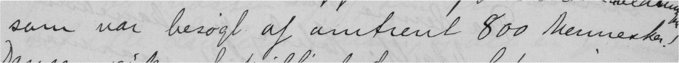som var besøgt af amtrent 800 Mennesker!
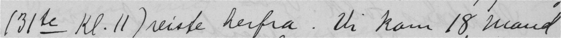(31te Kl. 11) reiste herfra. Vi kom 18 Mand
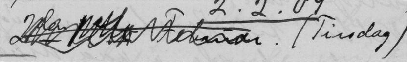. (Tirsdag)
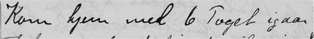Kom hjem med 6 Toget igaar
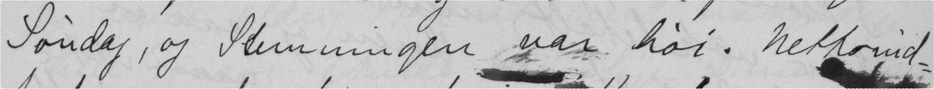Søndag, og Stemningen var høi. Nettoind-
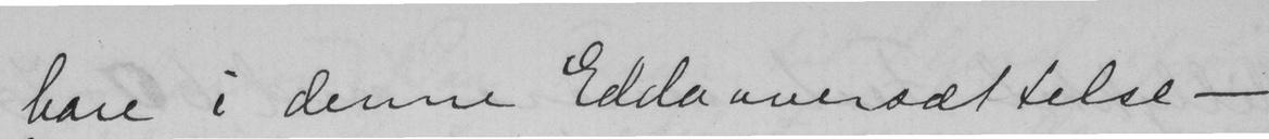bare i denne Edda oversættelse -
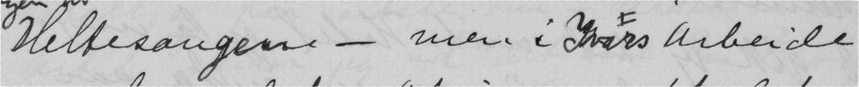Heltesangene - men i Ivars arbeide
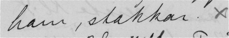ham, stakkar. x
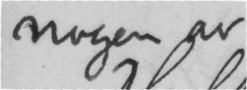nogen av
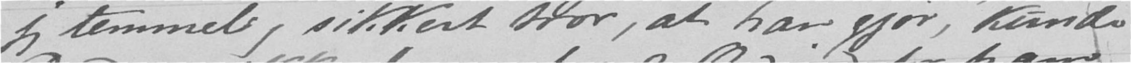jeg temmelig sikkert tror, at han gjør, kunde
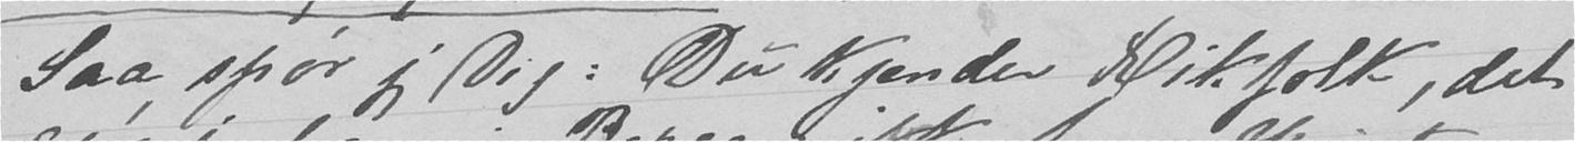Saa spør jeg Dig: Du kjender Rikfolk, det
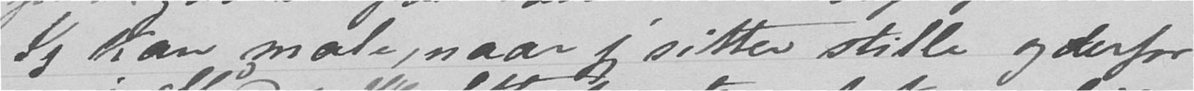Jeg kan male, naar jeg sitter stille og derfor
